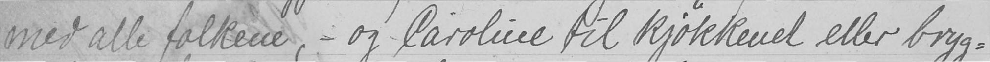med alle folkene, - og Caroline til kjøkkenet eller bryg-
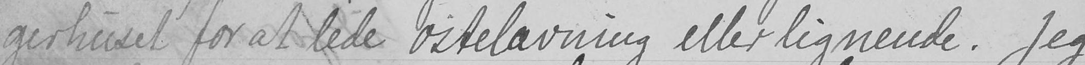gerhuset for at lede ostelavning eller lignende. Jeg
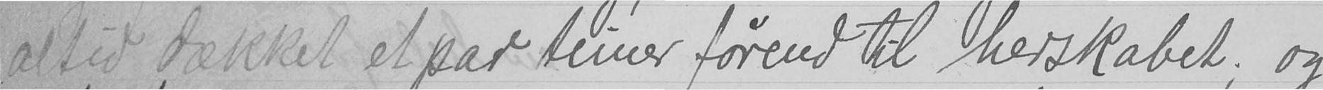altid dækket et par timer førend til herskabet; og
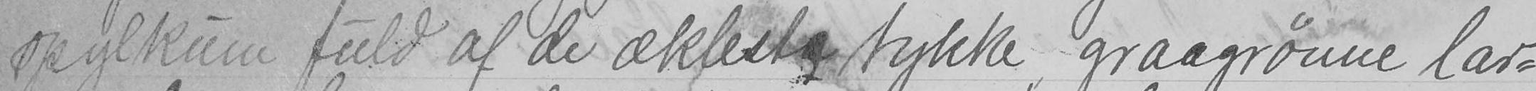spylkum fuld af de ækleste tykke, graagrønne lar-
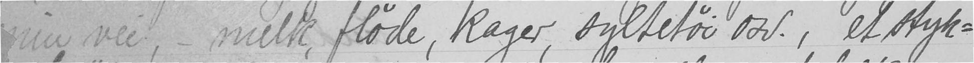min vei, - melk, fløde, kager, syltetøi osv., et styk-
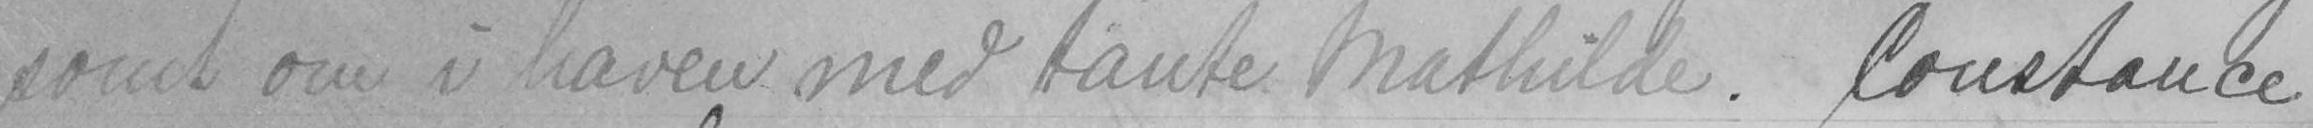somt om i haven med tante Mathilde. Constance
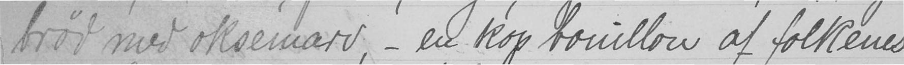brød med oksemarv, - en kop bouillon af folkenes
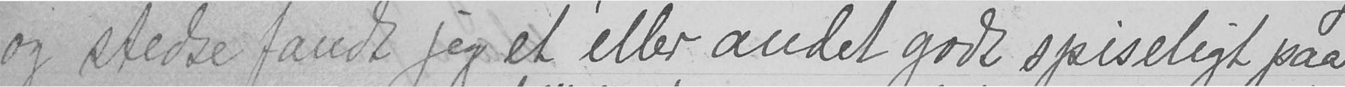og stedse fandt jeg et eller andet godt spiseligt paa
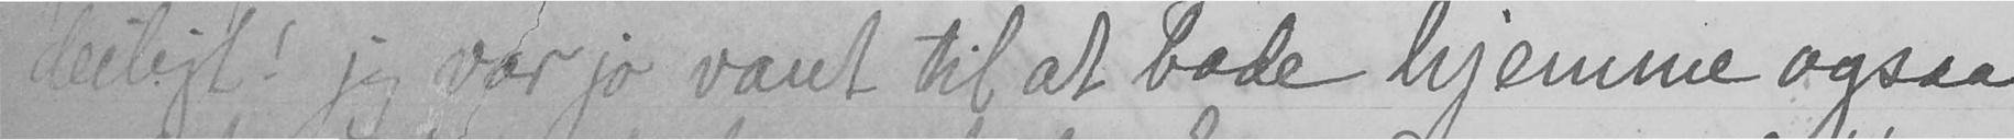deiligt! jeg var jo vant til at bade hjemme ogsaa
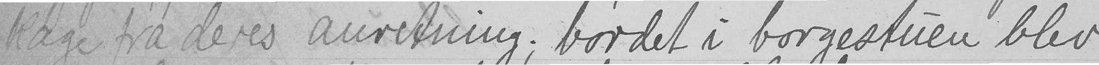kage fra deres anretning; bordet i borgestuen blev
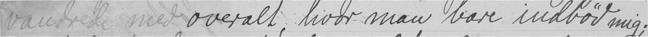vandrede med overalt, hvor man bare indbød mig;
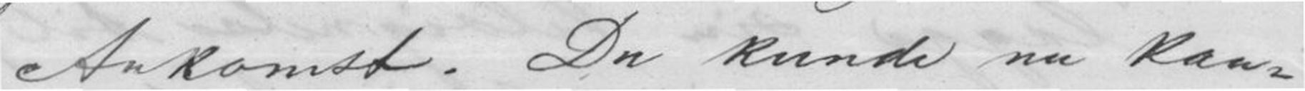Ankomst. Du kunde nu kan-
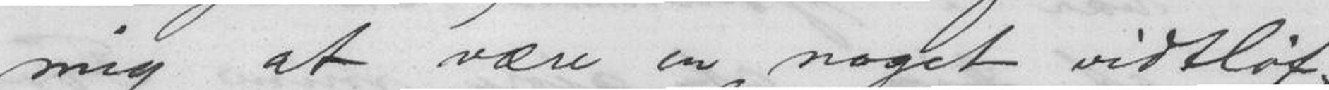mig at være en noget vidtløf-
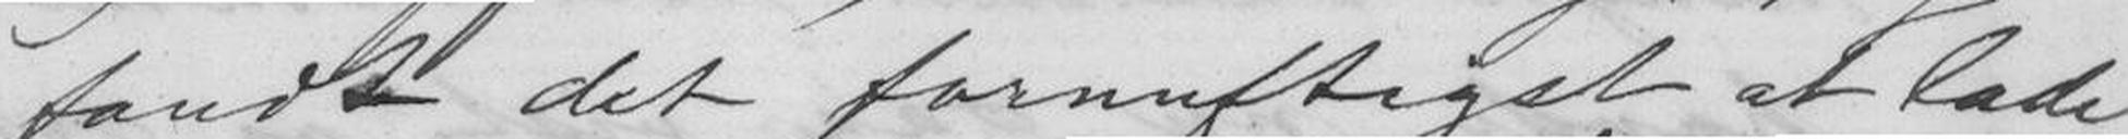fandt det fornuftigst at lade
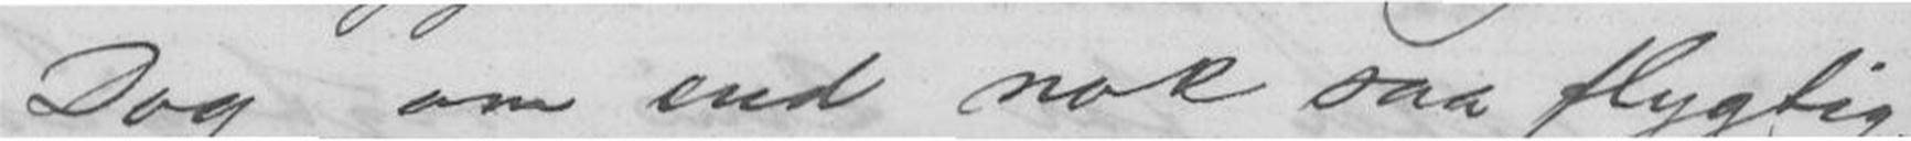Dog, om end nok saa flygtig,
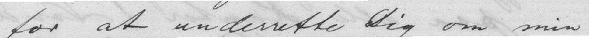for at underrette Dig om min
